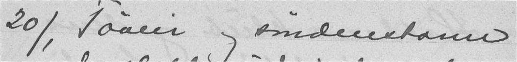20/1 Tøveir og søndenstorm
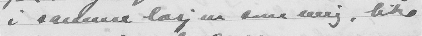i samme losjen som mig, like
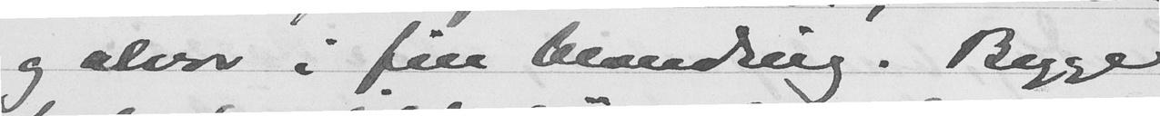og alvor i fin blanding. Begge
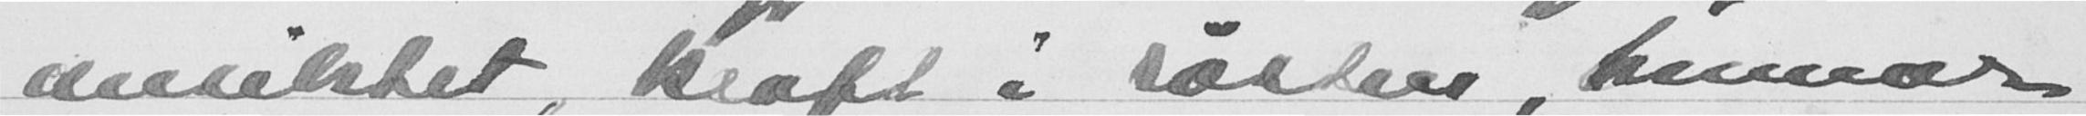ansiktet, kraft i røsten, hin
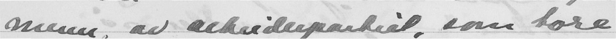menn, av arbeiderpartiet, som tøre
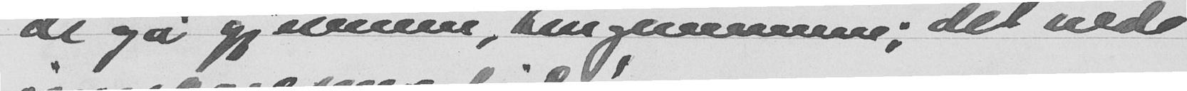de gå gjennom, bungeneen, det vilde
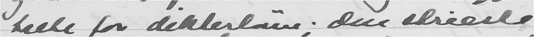talte for dikterlønn; den strieste
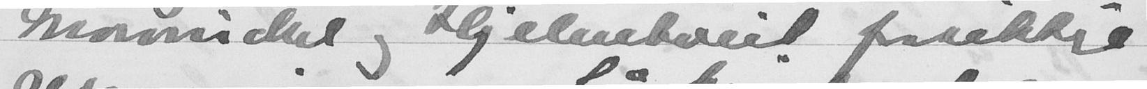Mowinckel og Hjelmtveit forsiktige
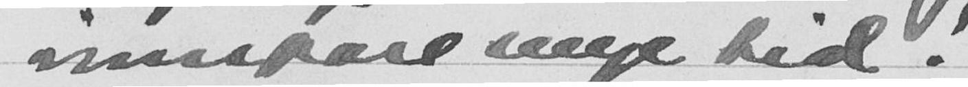innspare mye tid.
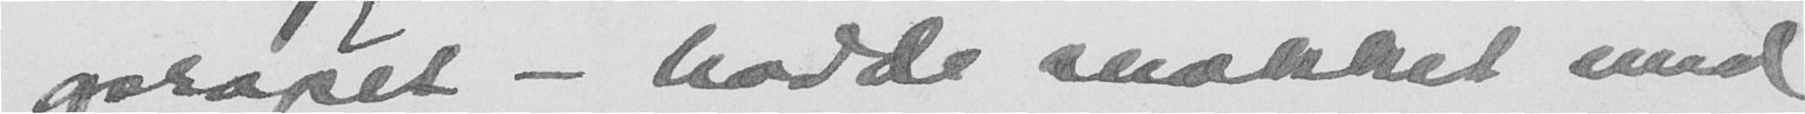opropet - hadde snakket med
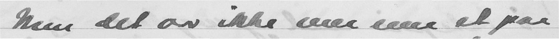Men det var ikke mer enn et par
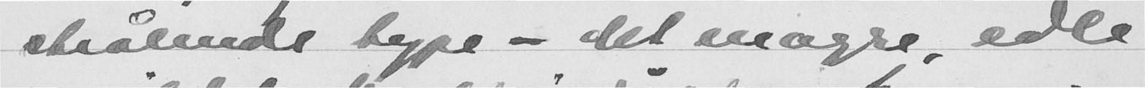strålende type - det magre, edle
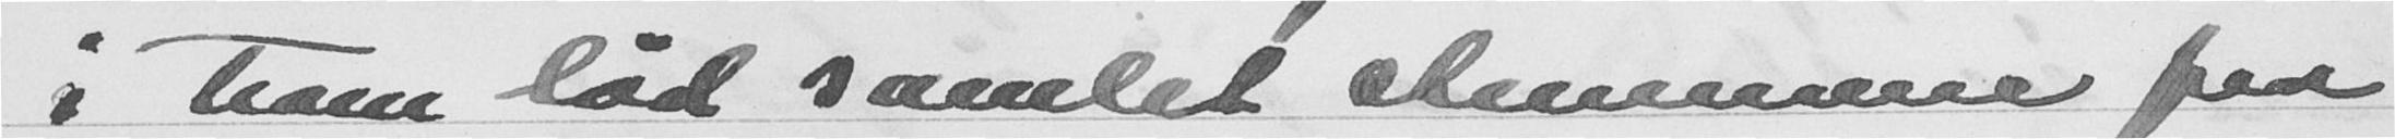i troen lød samlet stemmene for
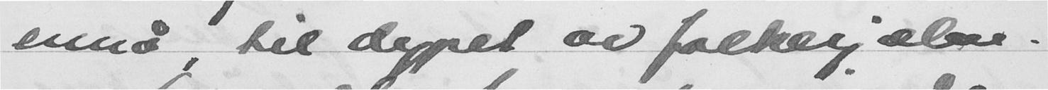ennå, til dypet av folkesjelen.
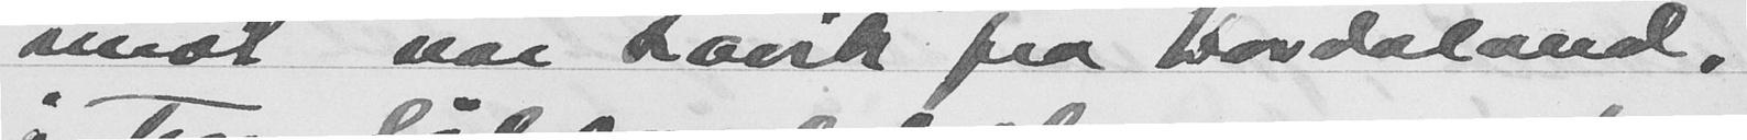imot var Hovik fra Hordaland,
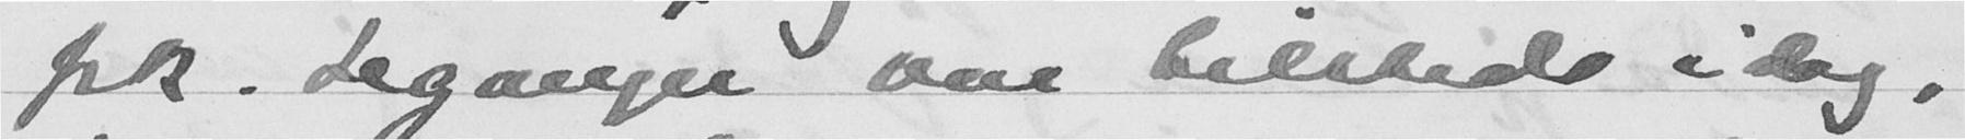frk. Leganger var tilstede i dag,
